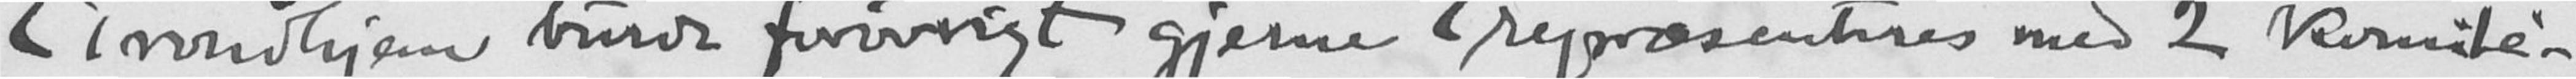Trondhjem burde forøvrigt gjerne repræsenteres med 2 komité-
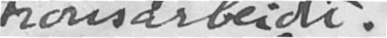tionsarbeidet.
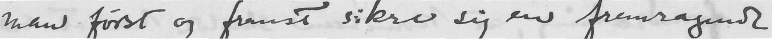man først og fremst sikre sig en fremragende
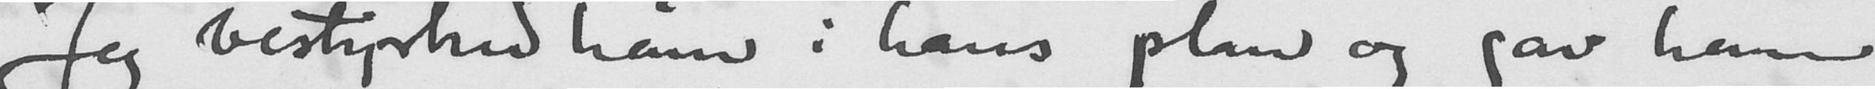Jeg bestyrked ham i hans plan og gav ham
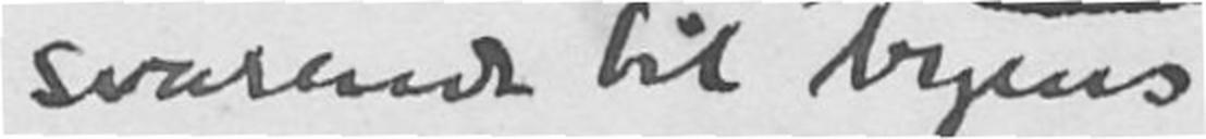svarende til byens
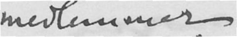medlemmer
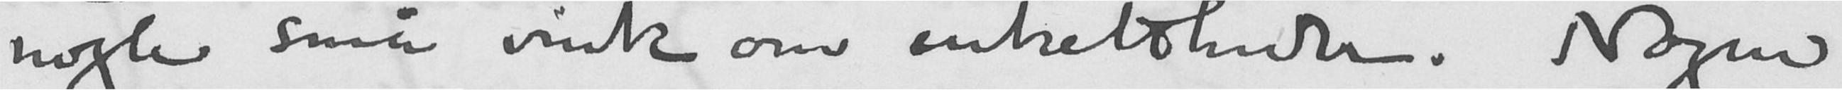nogle små vink om enkeltheder. Nogen
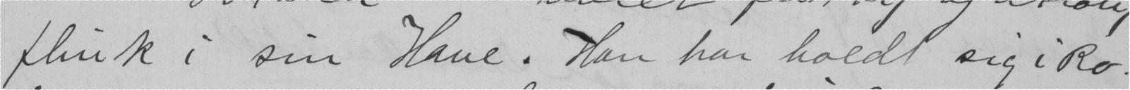flink i sin Have. Han har holdt sig i Ro.
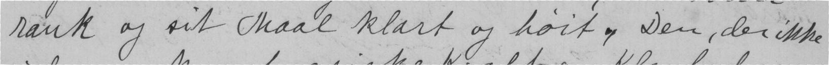rank og sit Maal klart og høit; Den, der ikke
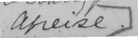Afreise.
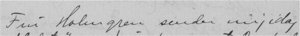Fru Holmgren sender mig idag
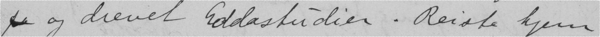og drevet Eddastudier. Reiste hjem
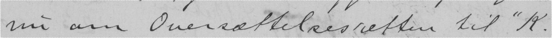nu om Oversættelsesretten til "K.
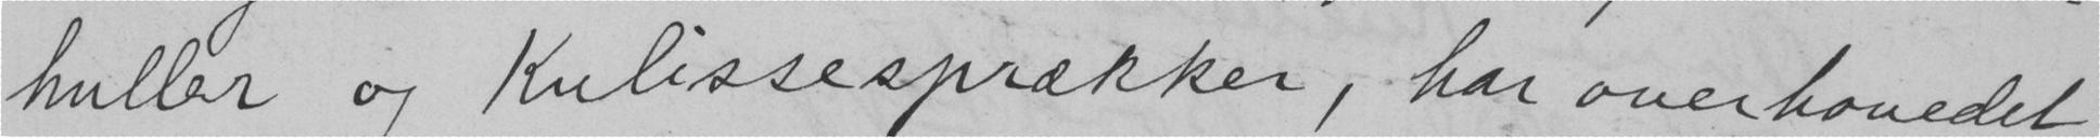huller og Kulissesprækker, har overhovedet
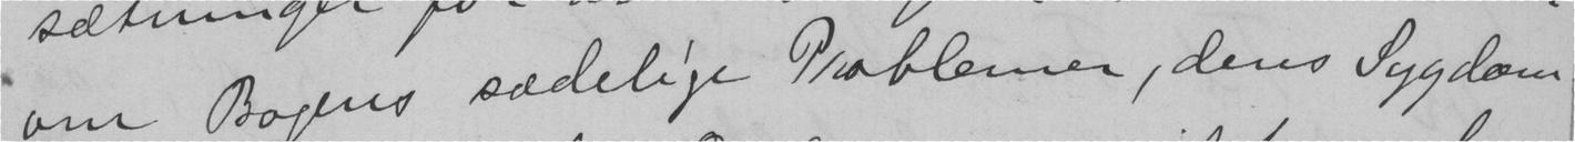om Bogens sædelige Prøblemner, dens sygdom.
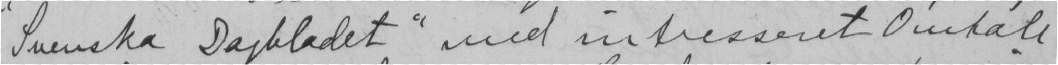"Svenska Dagbladet" med intresseret Omtale
